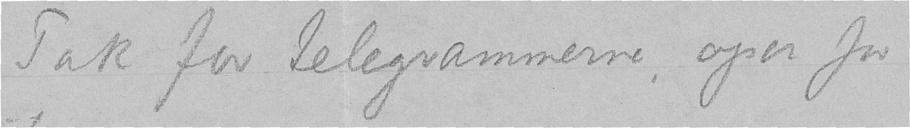Tak for telegrammerne, ogsaa for
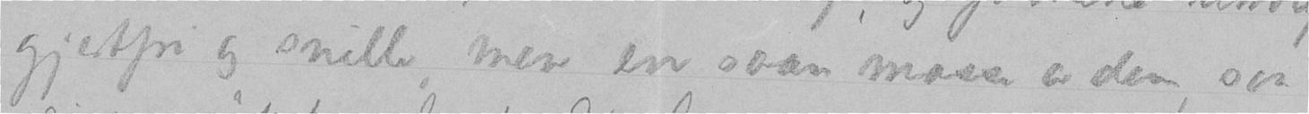gjestfri og snille, men en saan masse av dem, saa
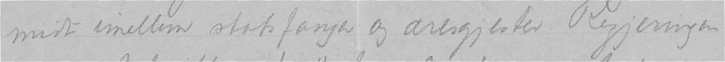midt imellem statsfanger og æresgjester. Regjeringen
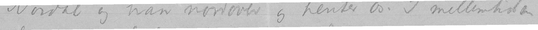Nordal og han nordover og henter os. I mellemtiden
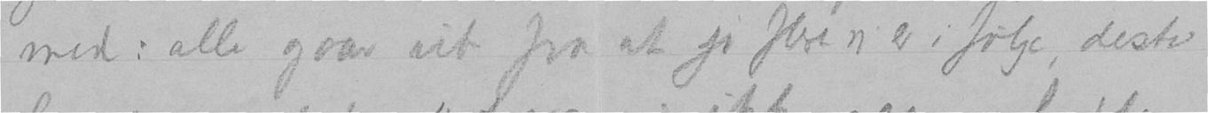med: alle gaar ut fra at jo flere vi er i følge, desto
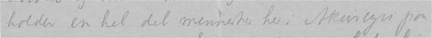holder en hel del mennesker her i Akureyri paa
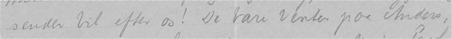sender bil efter os! De bare venter paa Anders,
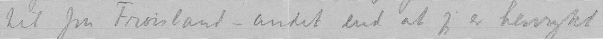til fra Frøisland – andet end at jeg er henrykt
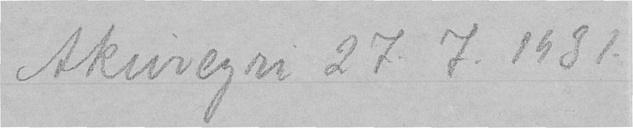Akureyri 27. 7. 1931.
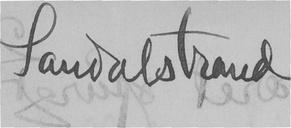Sandalstrand
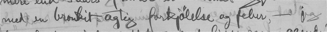med en bronkit-agtig forkjølelse og feber, - jeg
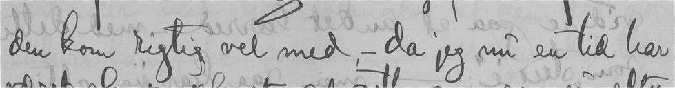den kom rigtig vel med, - da jeg nu en tid har
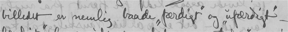billedet er nemlig baade "færdigt" og "ufærdigt" -
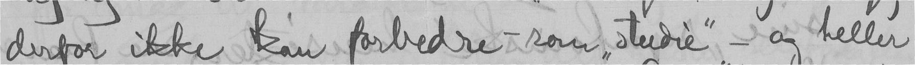derfor ikke kan forbedre - som "studie" - og heller
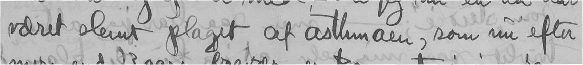været slemt plaget af asthmaen, som nu efter
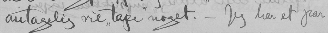antagelig vil "tape" noget. - Jeg har et par
